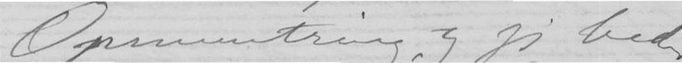Opmuntring, og jeg beder
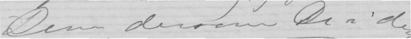Dem dersom De i disse
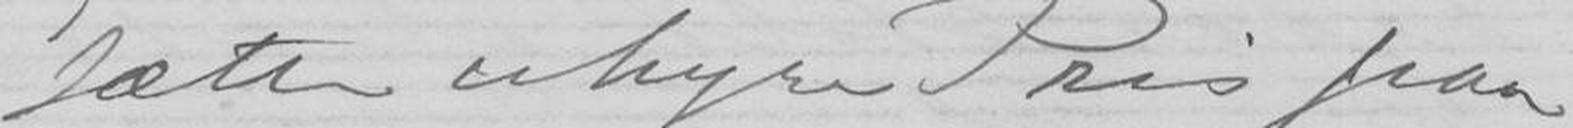sætte uhyre Pris paa
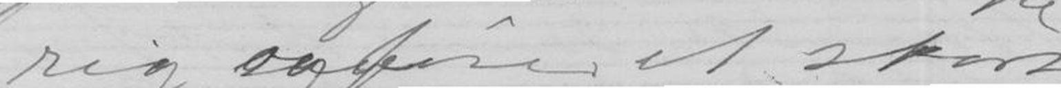rig og fører et stort
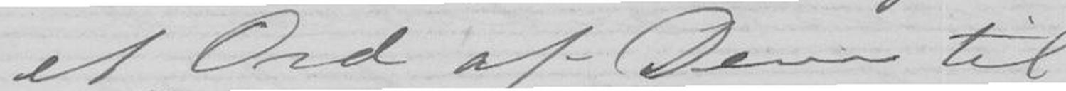et Ord af Dem til
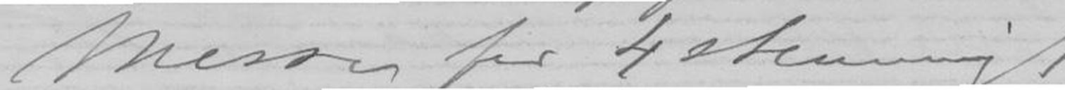Messe for 4 stemmigt
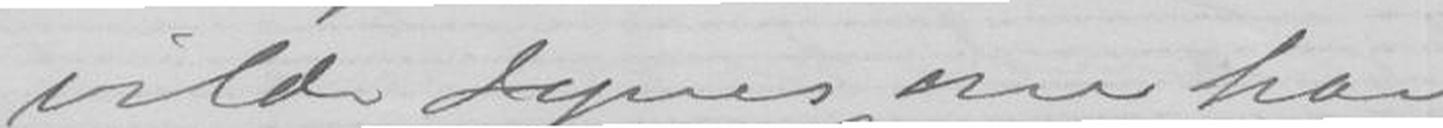vilde synes om ham
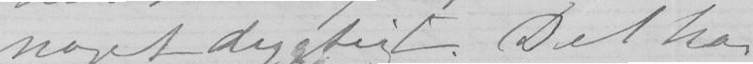noget dygtigt. Det har
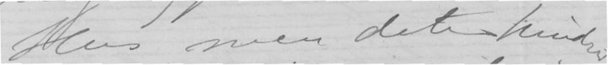Hus, men det hindrer
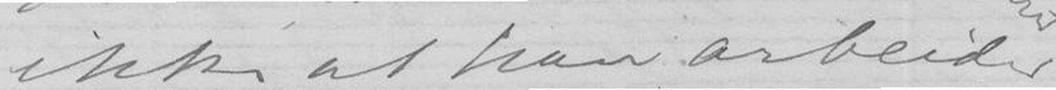ikke at han arbeider
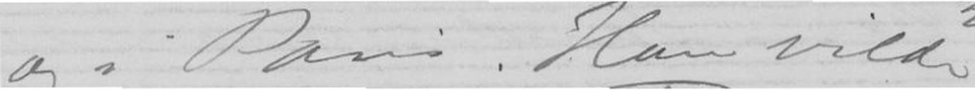og i Paris. Han vilde
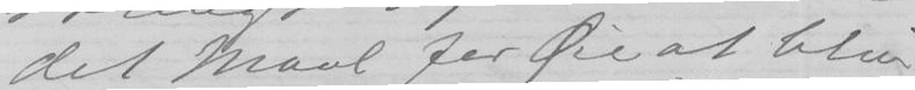det Maal for Øie at blive
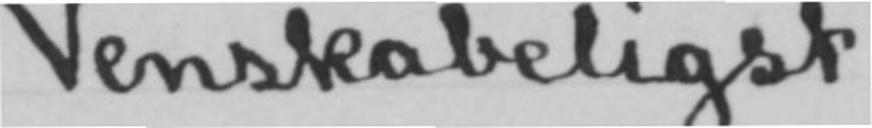Venskabeligst
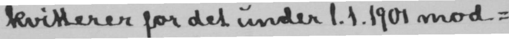kvitterer for det under 1.1.1901 mod-
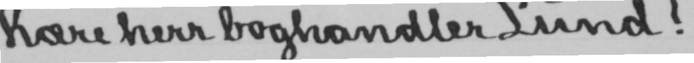Kære herr boghandler Lund!
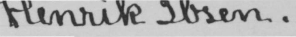Henrik Ibsen.
